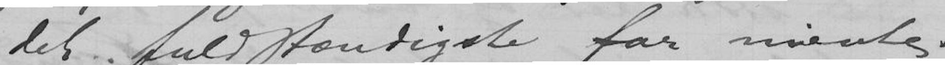det fuldstændigste for mintes.
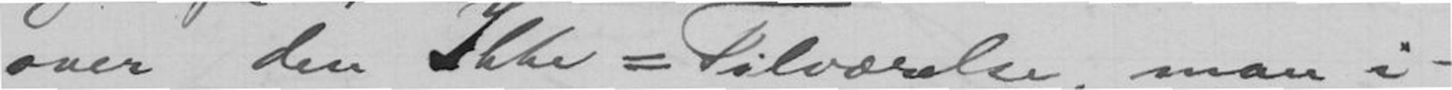over den Ikke=Tilværelse, man i-
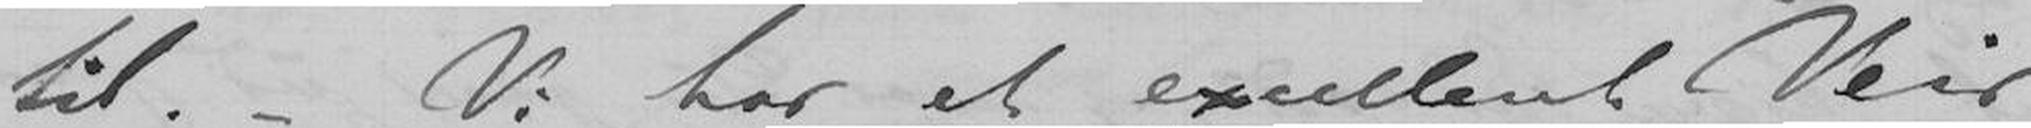til. - Vi har et exellent Veir
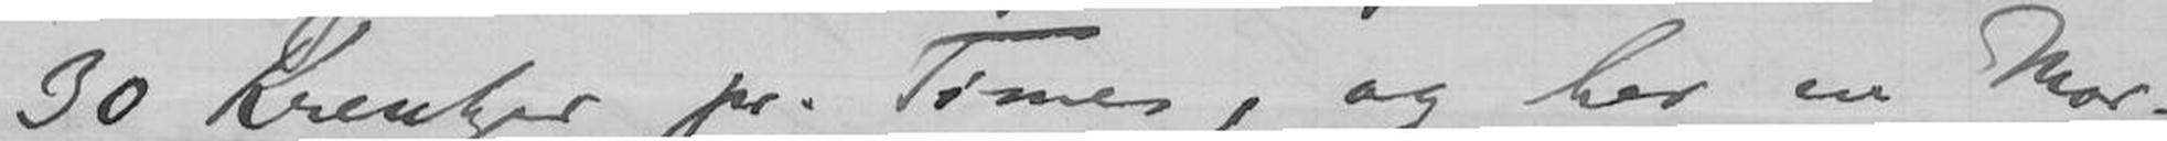30 Kreutzer pr. Time, og her en Mor-
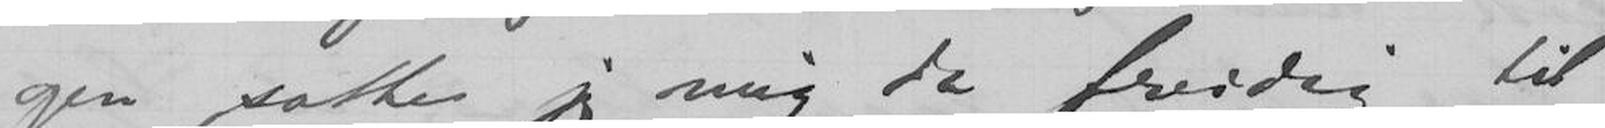gen satte jeg mig da freidig til
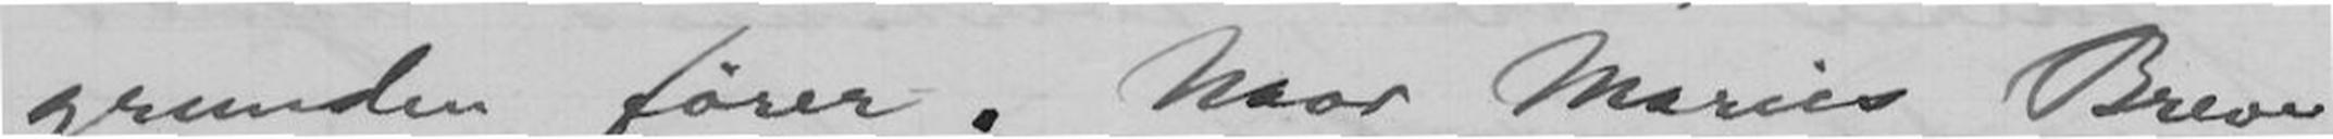grunden fører. Naar Maries Breve
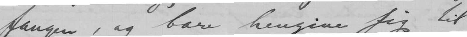fangen, og bare hengive sig til
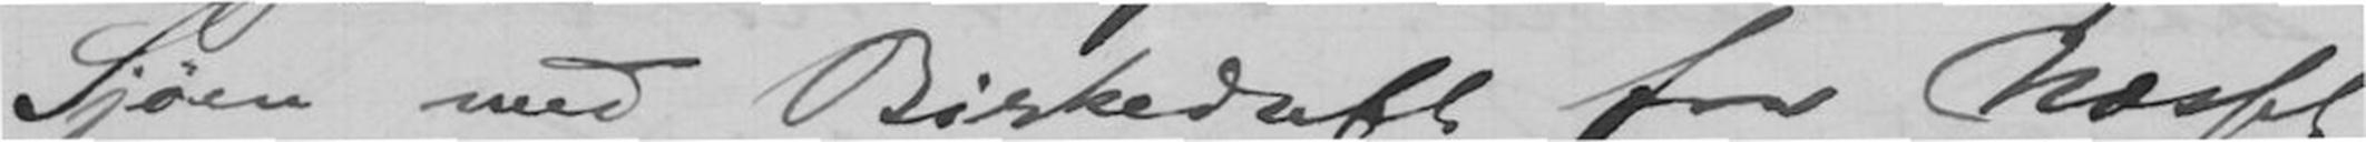Sjøen med Birkeduft fra Næsset
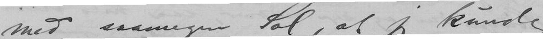med saamegen Sol, at jeg kunde
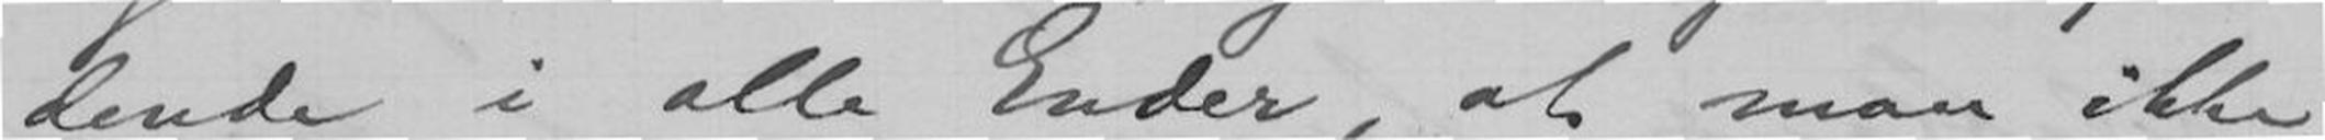dende i alle Ender, at man ikke
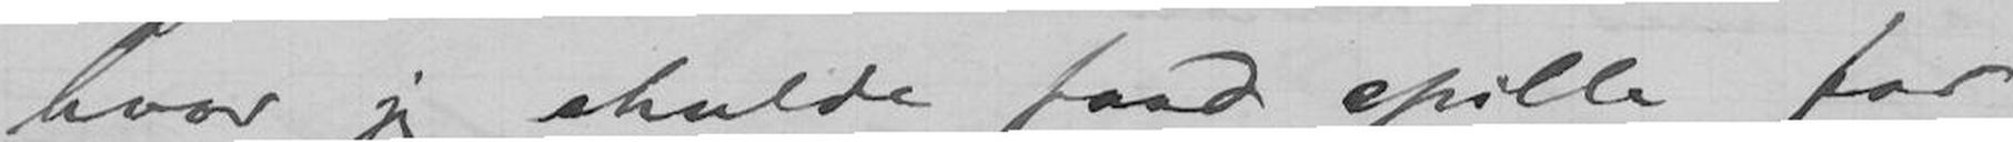hvor jeg skulde faad spille for
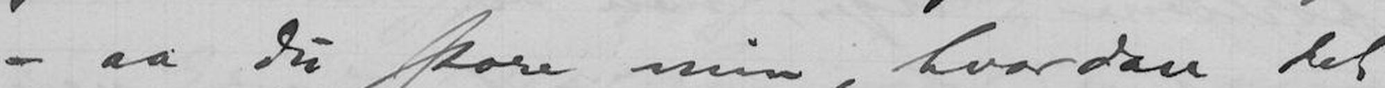- aa du store min, hvordan det
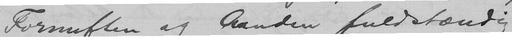Fornuften og Aanden fuldstændig
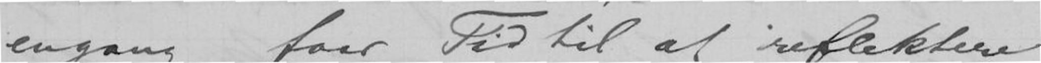engang faar Tid til at reflektere
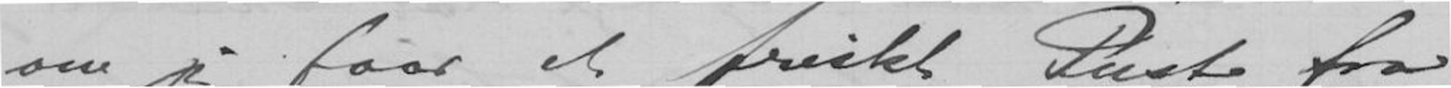om jeg faar et friskt Pust fra
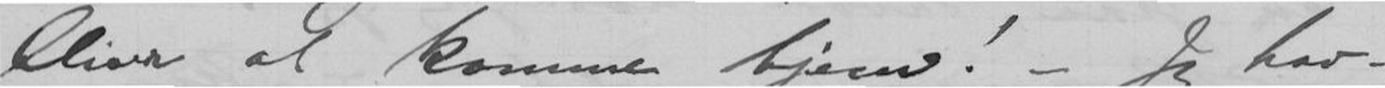bliver at komme hjem! - Jeg hav-
